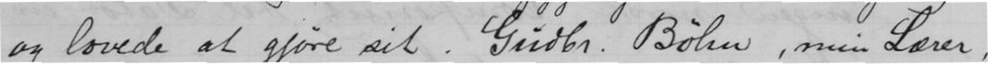og lovede at gjøre sit. Gudbr. Bøhn, min Lærer,
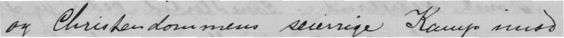og Christendommens seierrige Kamp imod
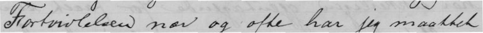Fortvivlelsen nær og ofte har jeg maattet
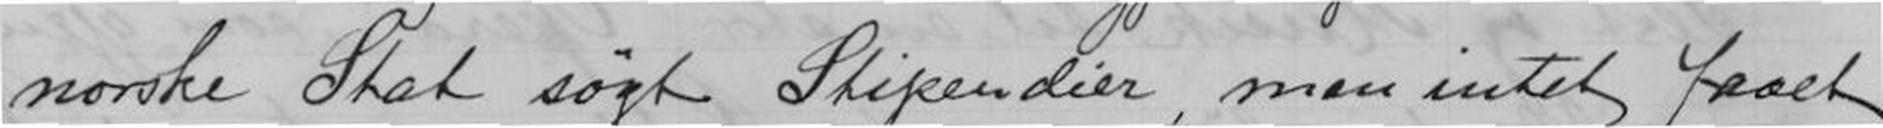norske Stat søgt Stipendier, men intet faaet
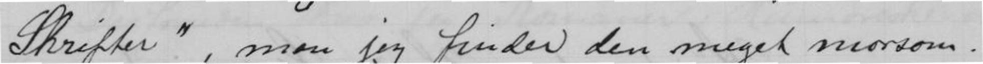Skrifter", men jeg finder den meget morsom.
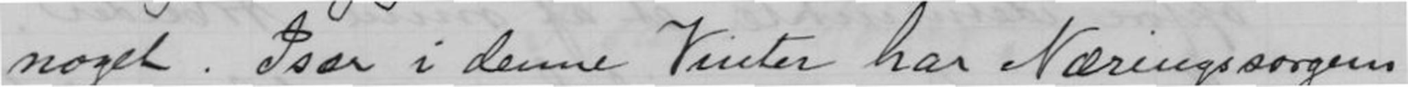noget. Især i denne Vinter har Næringssorgens
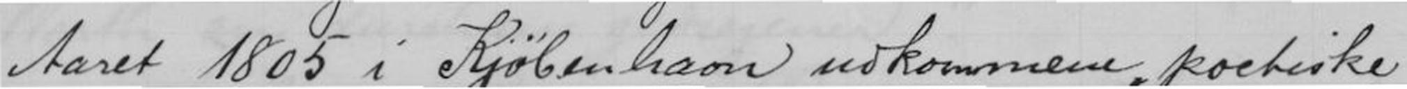Aaret 1805 i Kjøbenhavn udkommene "poetiske
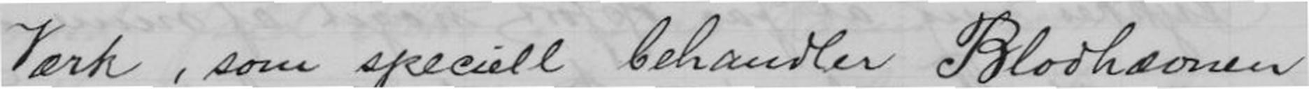Værk, som specielt behandler Blodhævnen
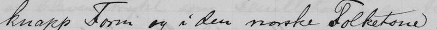knapp Form og i den norske Folketone.
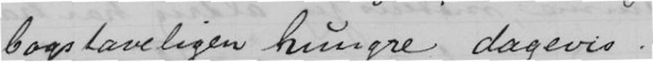bogstaveligen hungre dagevis.
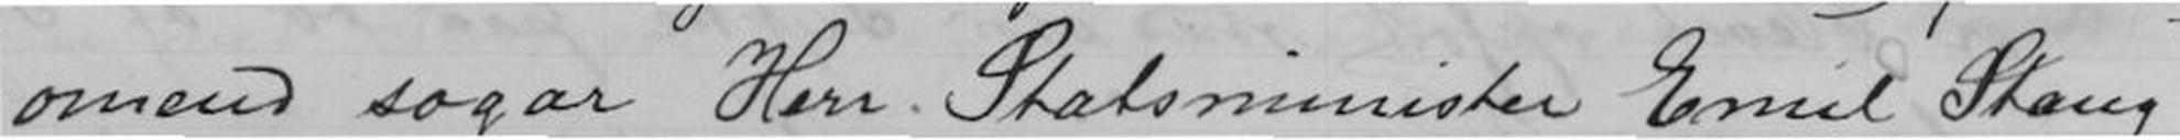omend sogar Herr Statsminister Emil Stang
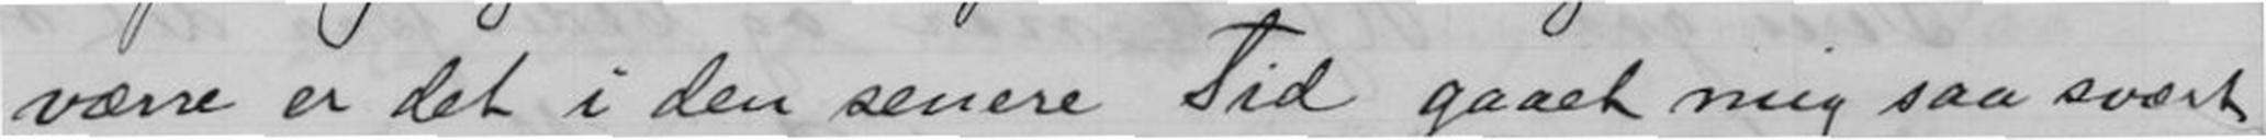værre er det i den senere Tid gaaet mig saa svært
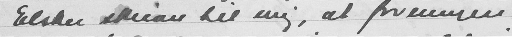Elster skriver til mig, at foreningen
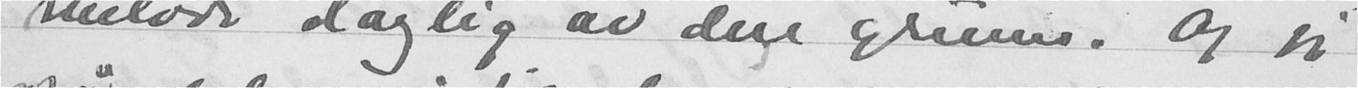melodi daglig av den grunn. Og jeg
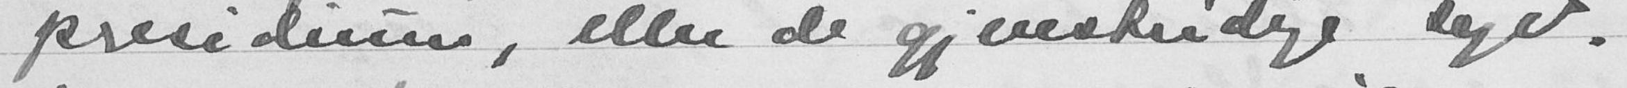presidium, eller de gjenstridige nye.
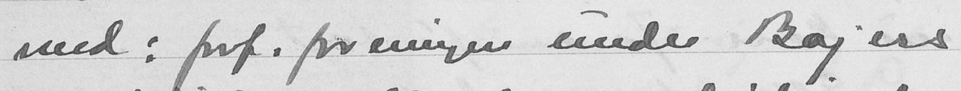med: forf. foreningen under Bojers
